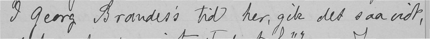I Georg Brandes's tid her, gik det saa vidt,
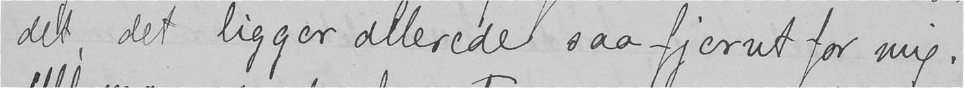det, det ligger allerede saa fjernt for mig.
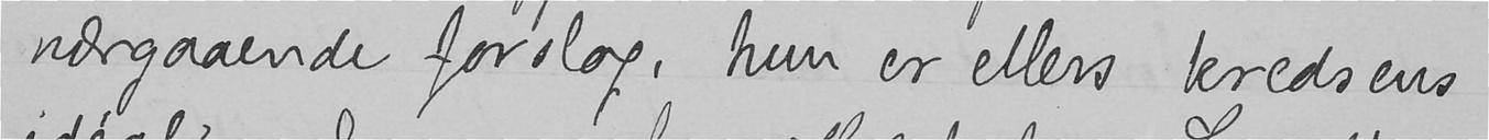nærgaaende forslag, hun er ellers kredsens
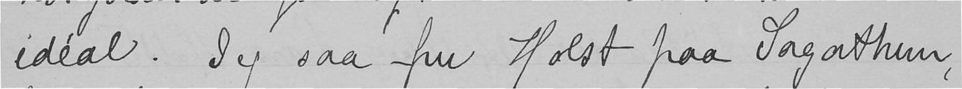idéal. Jeg saa fru Holst paa Sagathun,
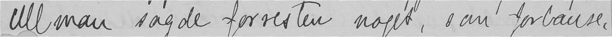Ullman sagde forresten noget, som forbause-
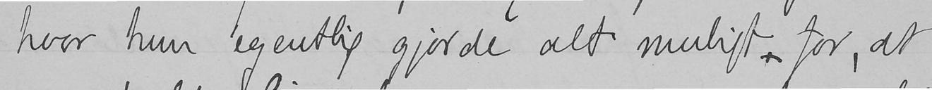hvor hun egentlig gjorde alt muligt for, at
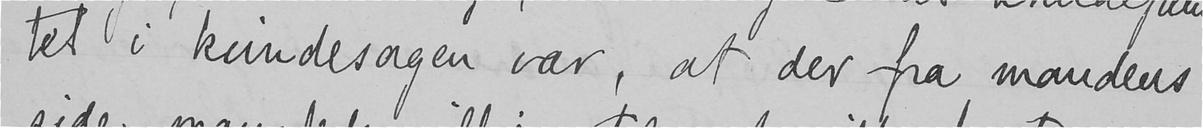tet i kvindesagen var, at der fra mandens
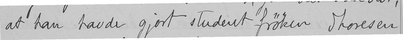at han havde gjort student frøken Thoresen
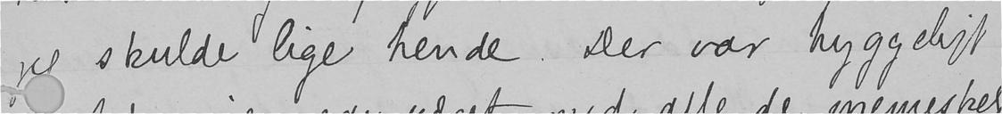jeg skulde lige hende. Der var hyggeligt
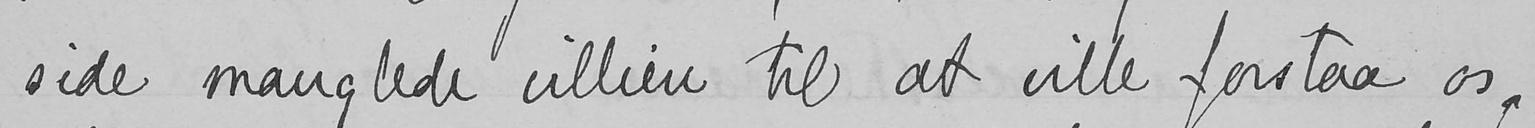side manglede villien til at ville forstaa os.
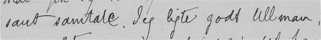sant samtale. Jeg ligte godt Ullman,
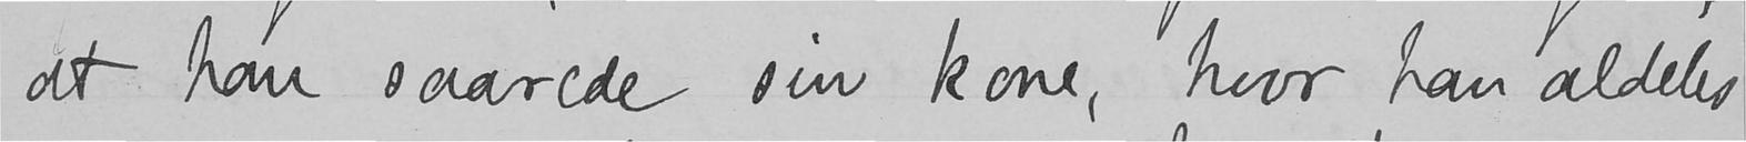at han saarede sin kone, hvor han aldeles
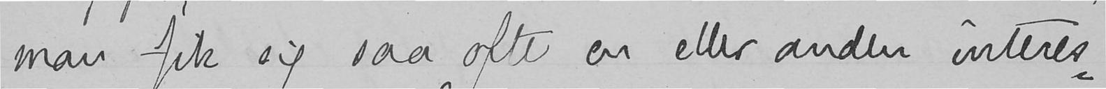man fik sig saa ofte en eller anden interes-
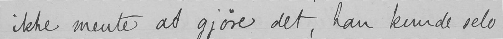ikke mente at gjøre det, han kunde selv
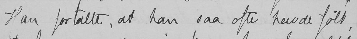Han fortalte, at han saa ofte havde følt,
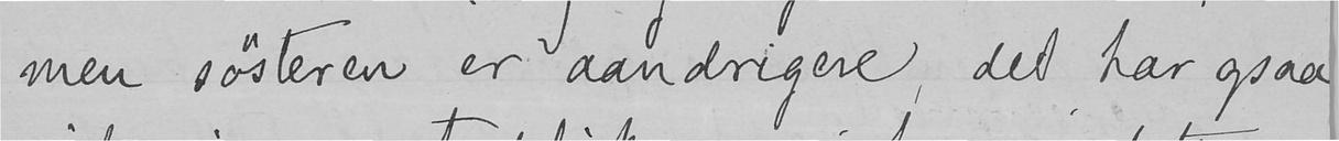men søsteren er aandrigere, det har ogsaa
